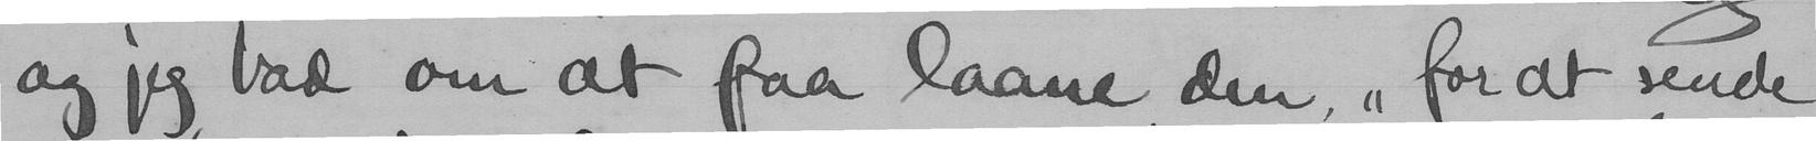og jeg bad om at faa laane den, "for at sende
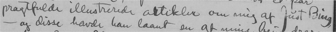pragtfulde illustrerede altikler om mig af Just Bing,
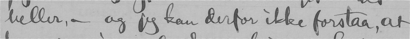heller, - og jeg kan derfor ikke forstaa, at
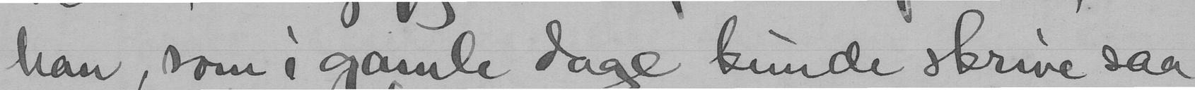han, som i gamle dage kunde skrive saa
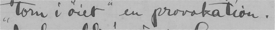"torn i øiet" en provokation.
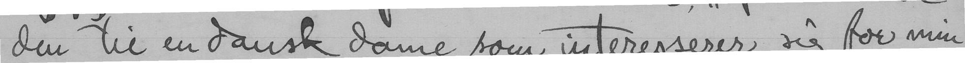den til en dansk dame som interesserer sig for min
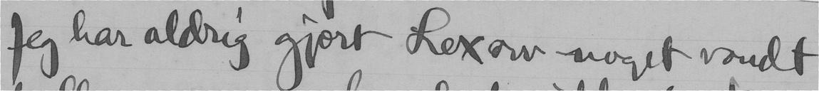Jeg har aldrig gjort Lexow noget vondt
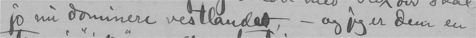jo nu dominere vestlandet, - og jeg er dem en
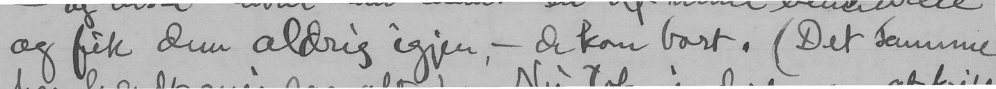og fik dem aldrig igjen, - de kom bort. (Det samme
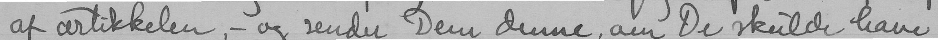af artikkelen, - og sender Dem denne, om De skulde have
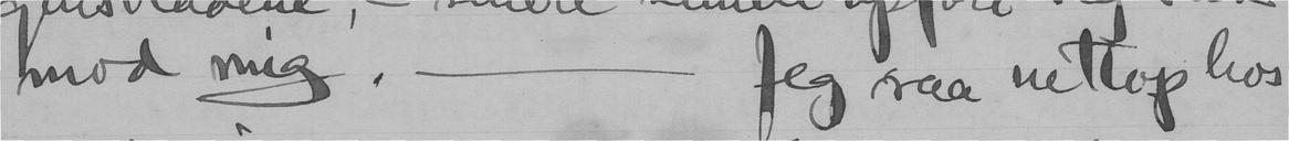mod mig . - Jeg saa nettop hos
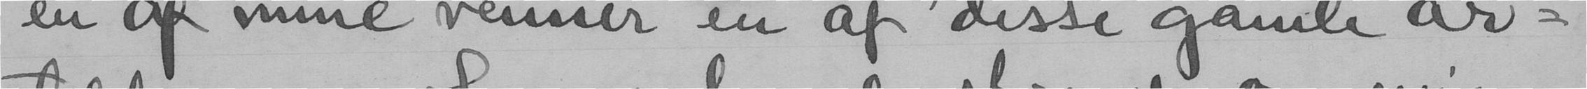en af mine venner en af disse gamle ar-
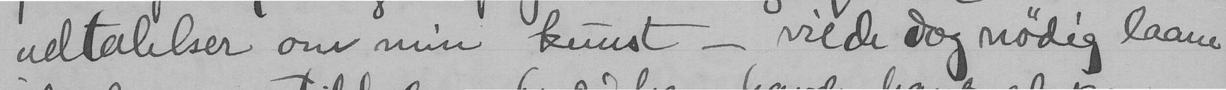udtalelser om min kunst - vilde dog nødig laane
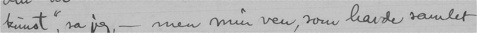kunst", sa jeg, - men min ven, som havde samlet
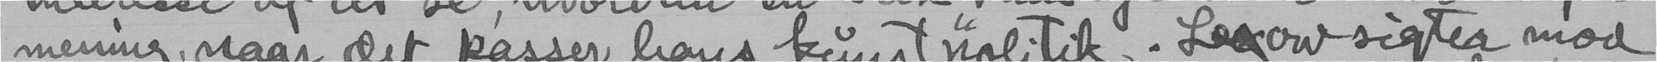mening, naar det passer hans kunstpolitik. Lexow sigter mod
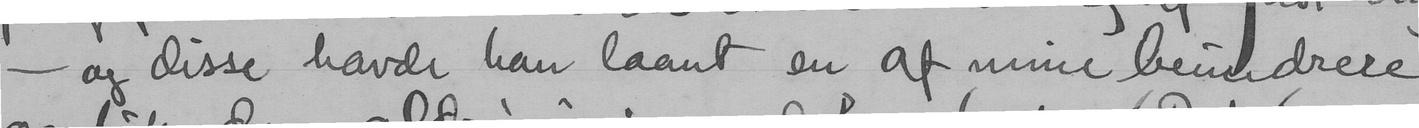og disse havde han laant en af mine beundrere
